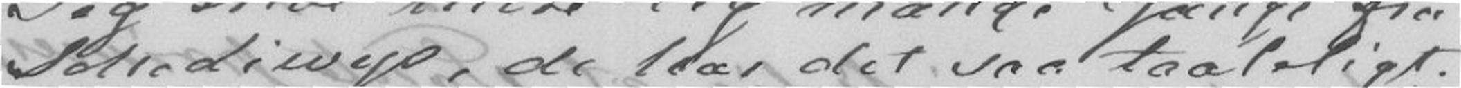Schediwys, de har det saa taaleligt.
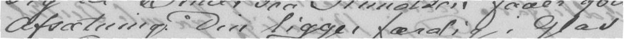Afsetning. Din ligger færdig i Glas
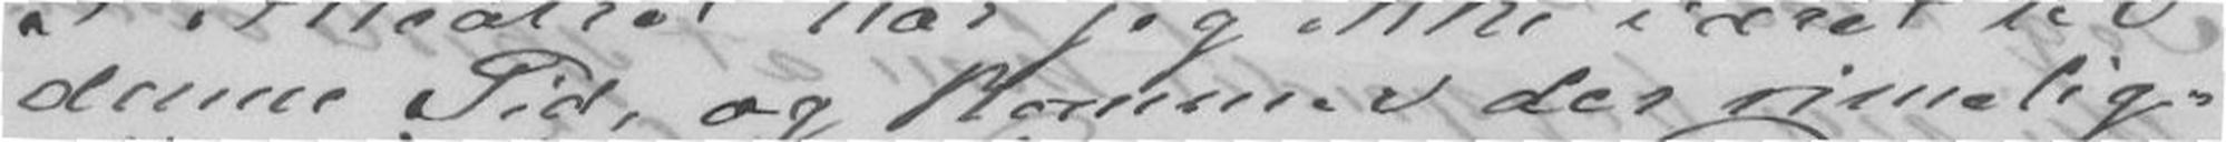denne Tid, og kommer der rimelig-
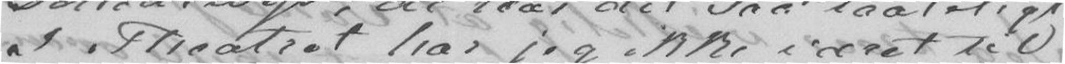I Theatret har jeg ikke været til
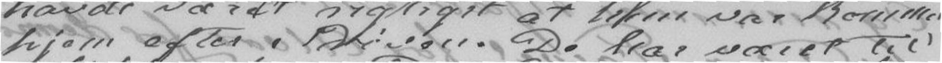hjem efter Prøven. De har været til
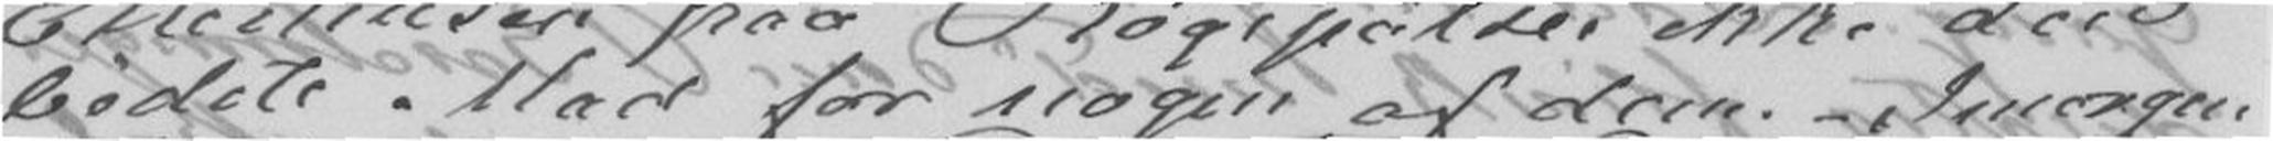bedste Mad for nogen af dem. - Imorgen
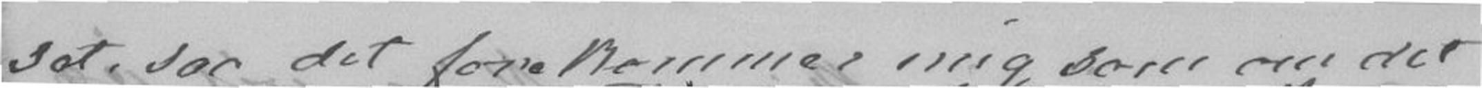sot, saa det forekommer mig som om det
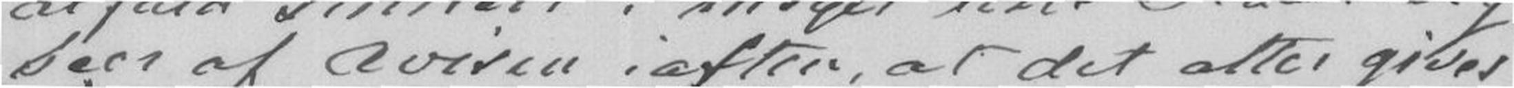seer af Avisen iaften, at det atter gives
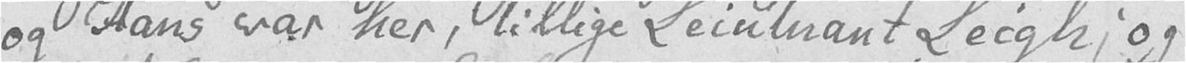og Hans var her, tillige Leiutnant Leigh, og
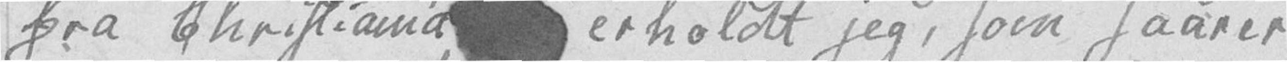fra Christiania  erholdt jeg, som saarer
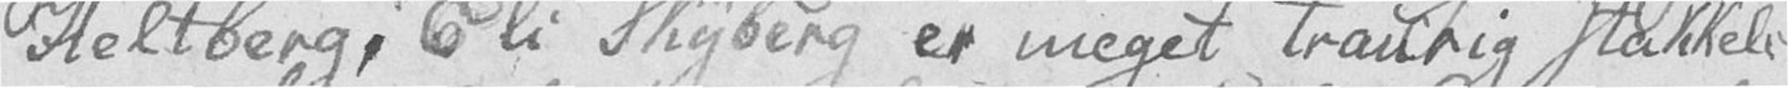Heltberg, Eli Skyberg er meget traurig stakkels
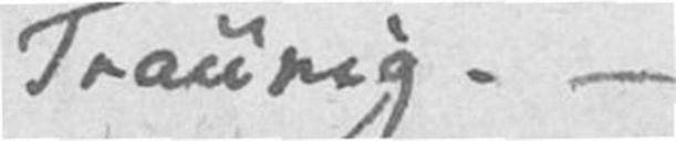Traurig. –
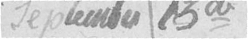September 13de
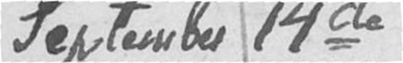September 14de
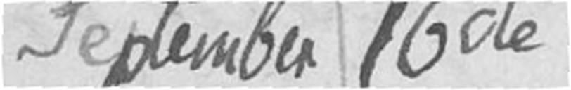September 16de
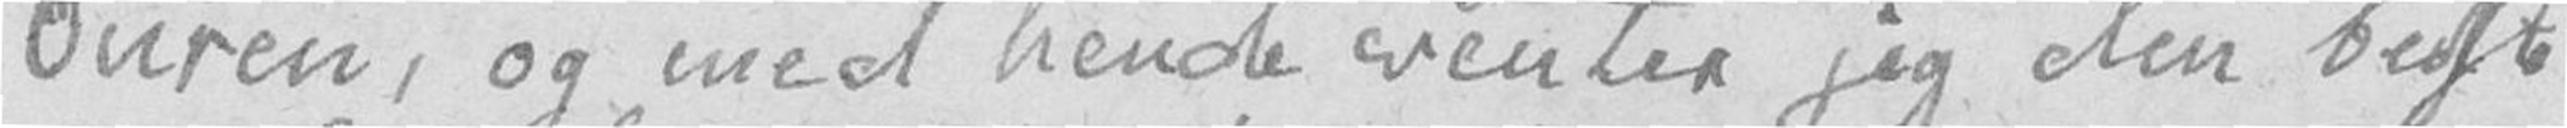Ouren, og med hende venter jeg den bedste
